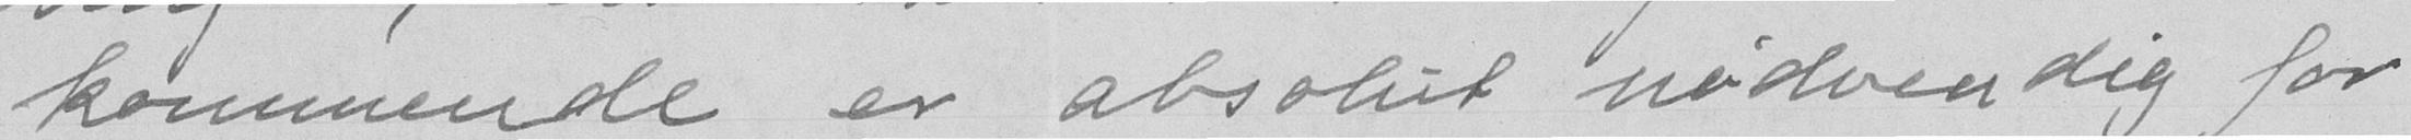kommende er absolut nødvendig for
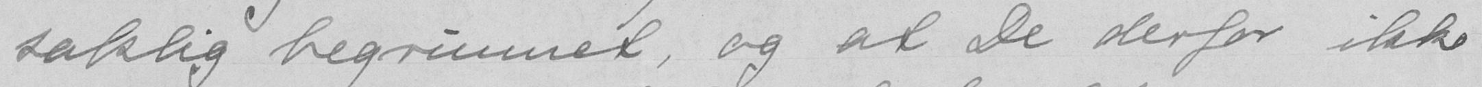saklig begrunnet, og at De derfor ikke
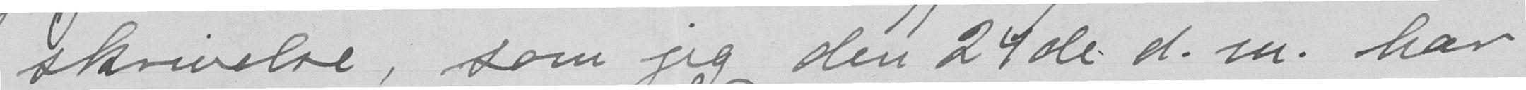skrivelse; som jeg den 24de d. m. har
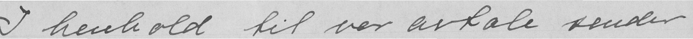I henhold til vor avtale sender
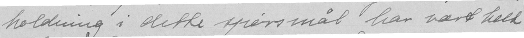holdning i dette spørsmål har være helt
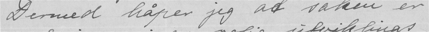Dermed håper jeg at saken er
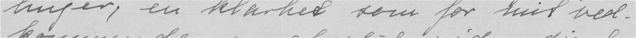linjer, en klarhed som for mit ved-
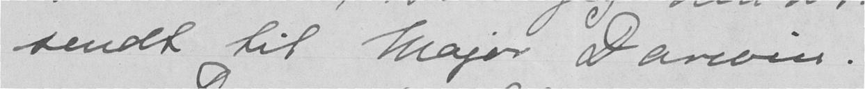sendt til Major Darwin.
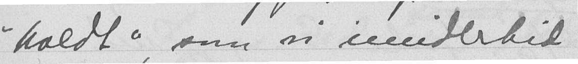"holdt", som vi imidlertid
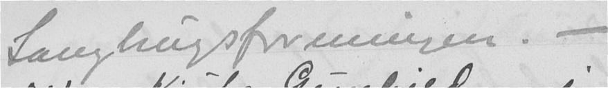Saugbrugsforeningen. -
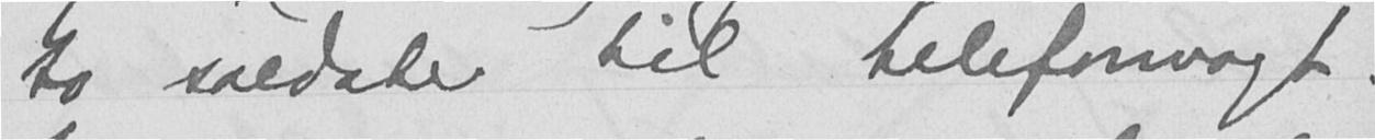to soldater til telefonvagt.
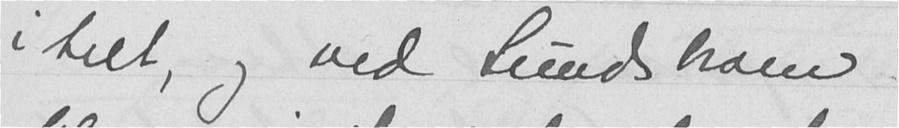i telt, og ved Sundsbroen
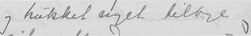og trukket noget tilbage og
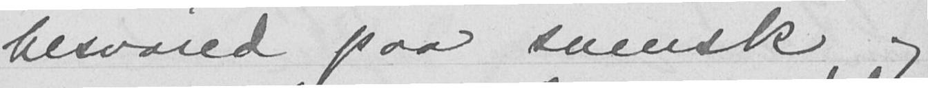besvared paa svensk og
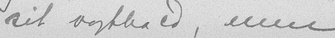sit vagthold, men
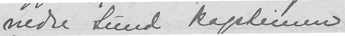nedre Sund kapteinen
